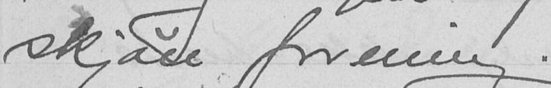skjøn forening.
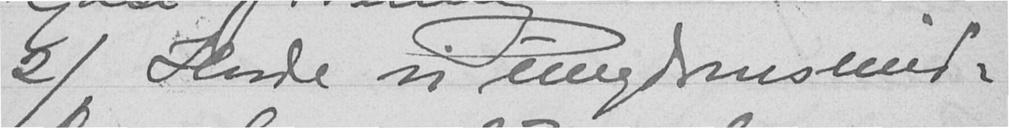2/1 Havde vi ungdomsmid.
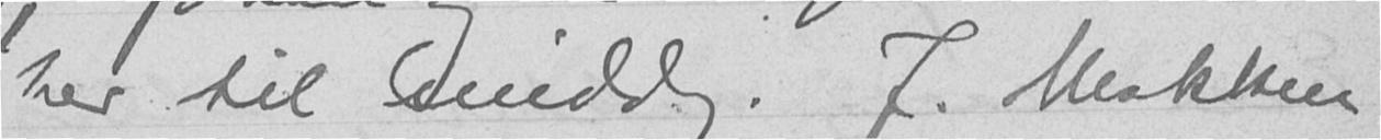her til middag. J. Makken
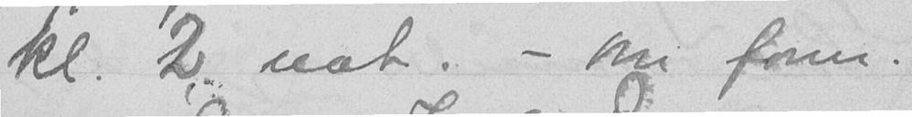kl. 2 nat. - Om form.
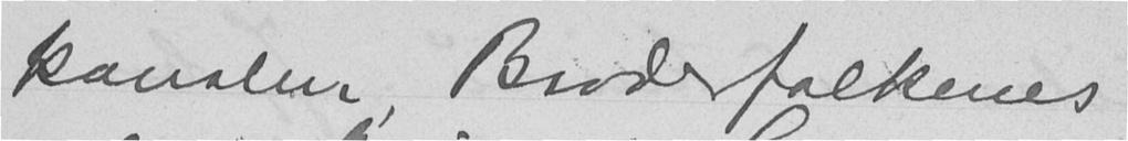kanalen, Broderfolkenes
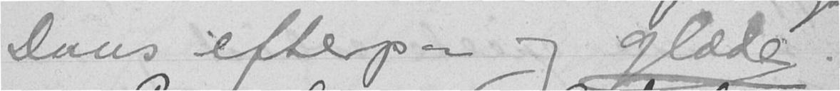Dans efterpaa og glæde.
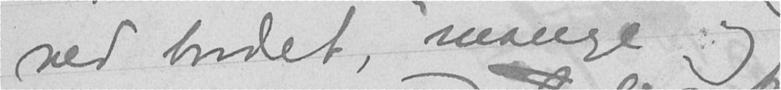ved bordet, mange og
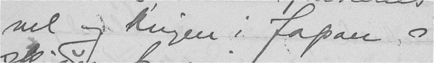vel og krigen i Japan i
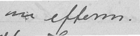om efterm.
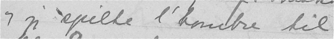og jeg spilte l'hombre til
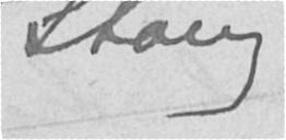Stang
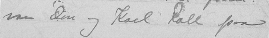var Don og Karl Roll paa
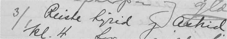3/1 Reiste Sigrid og Astrid
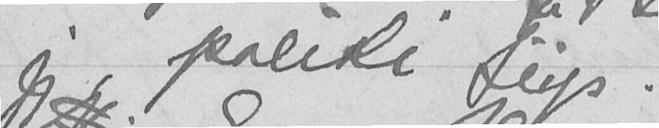jeg, politi Seip.
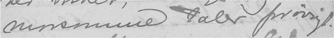morsomme taler forøvrigt.
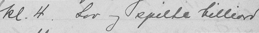kl. 4. Sov og spilte billiard
